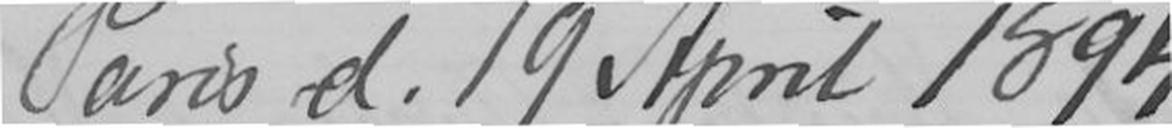Paris d. 19 April 1894.
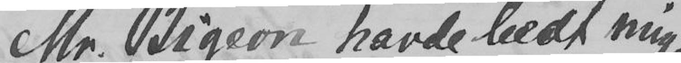Mr Bigeon havde bedet mig,
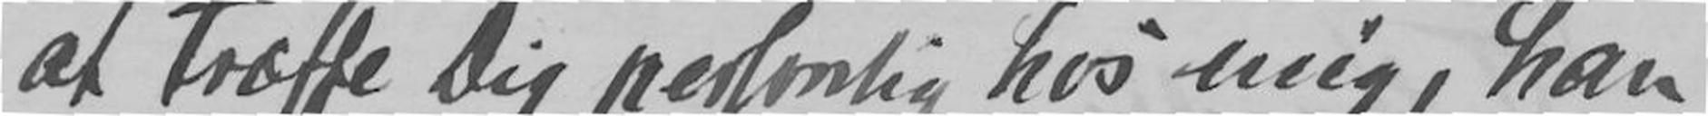at træffe Dig personlig hos mig, han
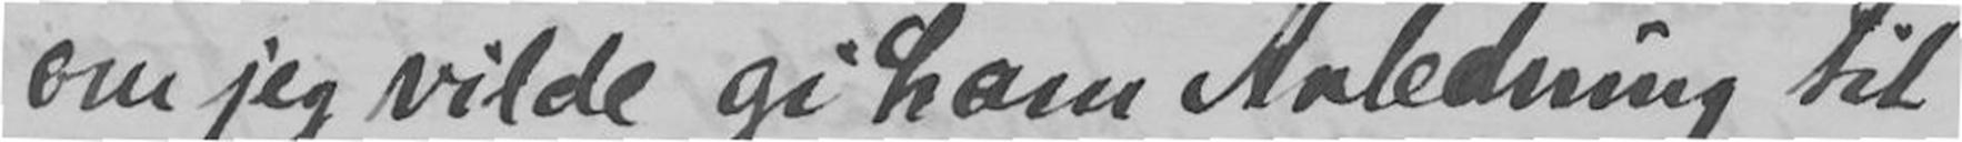om jeg vilde gi ham Anledning til
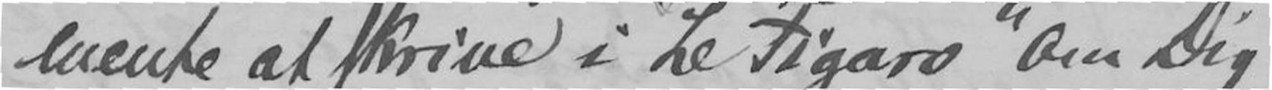mente at skrive i "Le Figaro" om Dig
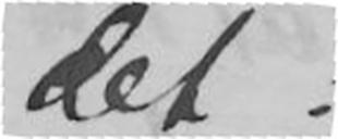det!
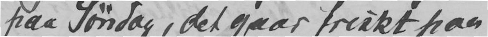paa Søndag, det gaar friskt paa
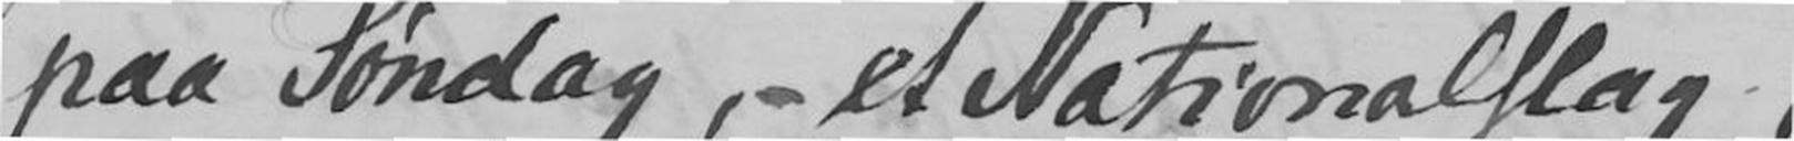paa Søndag, - et Nationalflag,
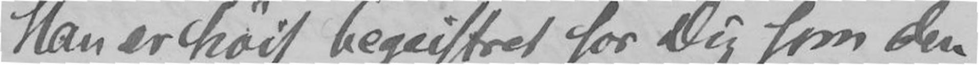Han er høit begeistret for Dig som den
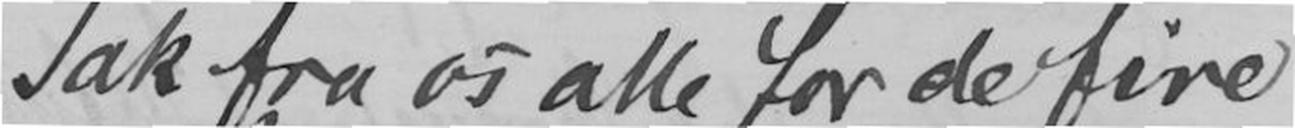Tak fra os alle for de fire
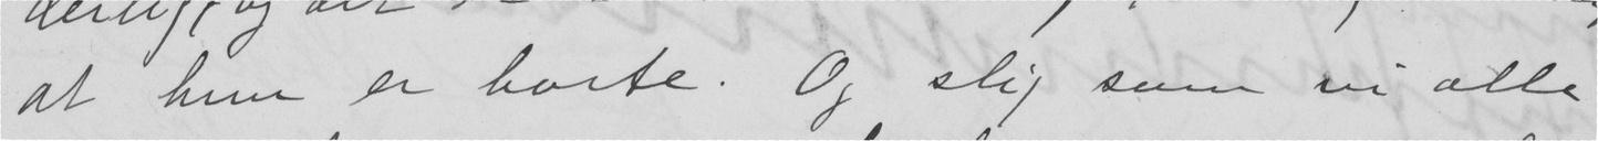at hun er borte. Og slig som vi alle
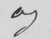og
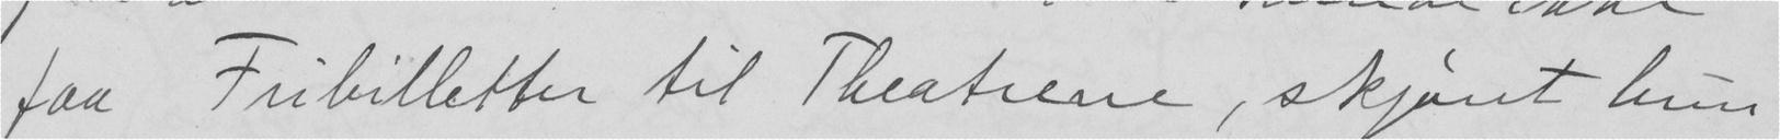faa Fribilletter til Theatrene, skjønt hun
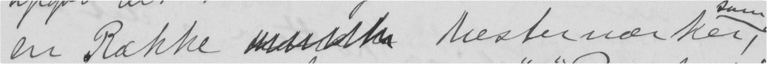en række  Mesterværker,
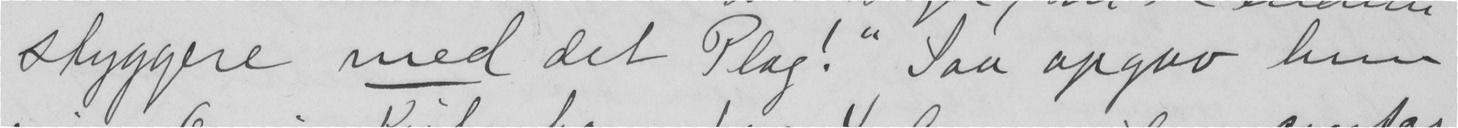styggere med det Plag!" Saa opgav hun
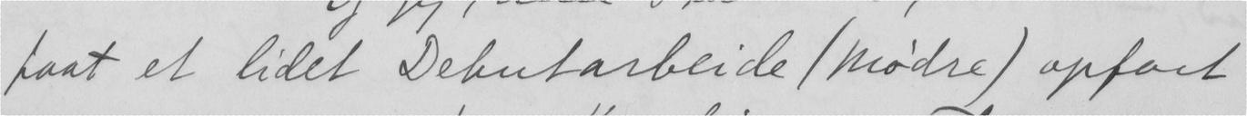faat et lidet Debutarbeide (Mødre) opført
